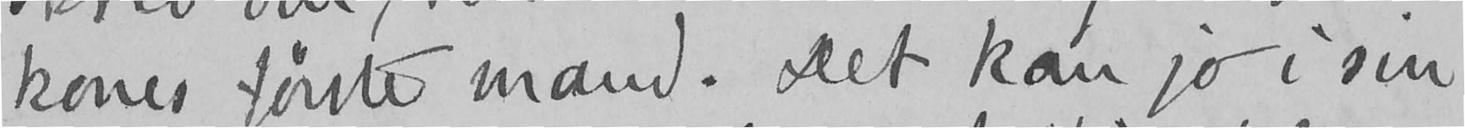kones første mand. Det kan jo i sin
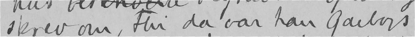skrev om, thi da var han Garborgs
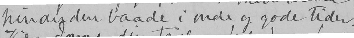hinanden baade i onde og gode tider.
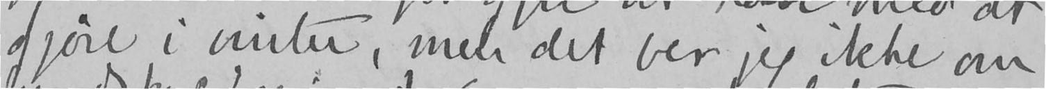gjøre i vinter, men det ber jeg ikke om
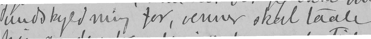undskyldning for, venner skal taale
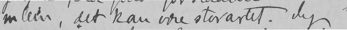mlem, det kan være storartet. Jeg
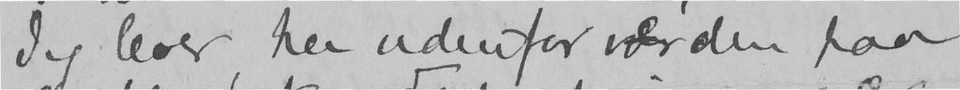Jeg lever her udenfor værden paa
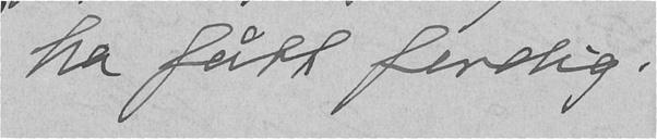fa fått ferdig!
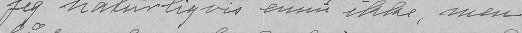jeg naturligvis ennu ikke men
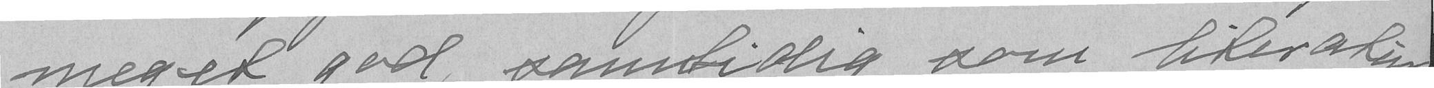meget god, samtidig som literatur
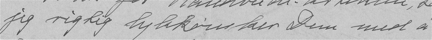sig rigtig lykkønsker Dem med å
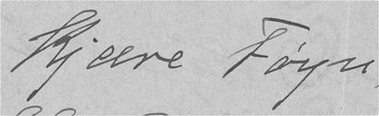Kjære Føyn,
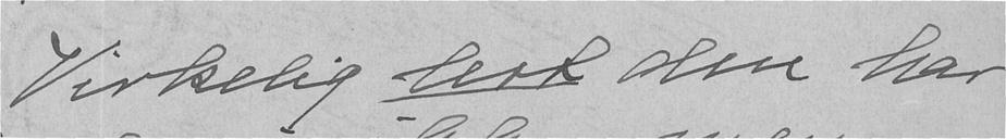Virkelig lest den har
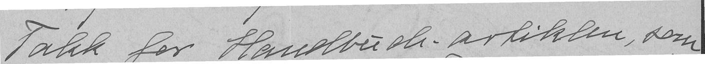Takk for Handbuch-artiklen, som
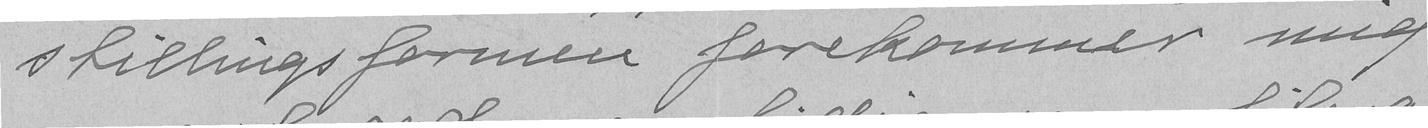stillingsformen forekommer meg
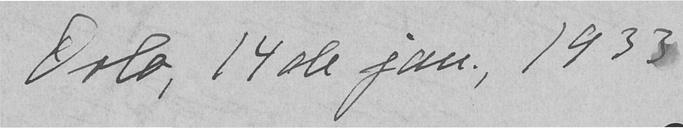Oslo, 14de jan., 1933
